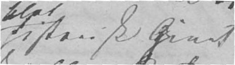historisk Givet
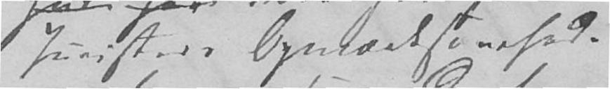Juristers Opmærksomhed.
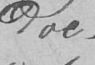Doc-
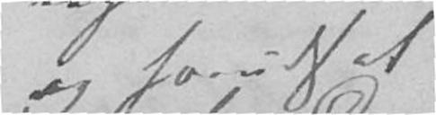og forudsat
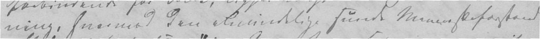ning, hvormed den almindelige sunde Menneskeforstand
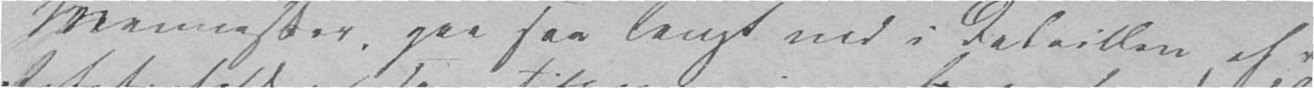Mennesker, gaa saa langt ind i Detaillen af
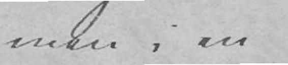man i en
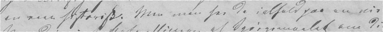en reen historisk. Men man har da ialfald paa en vis
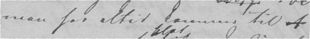man her altid kommer til at
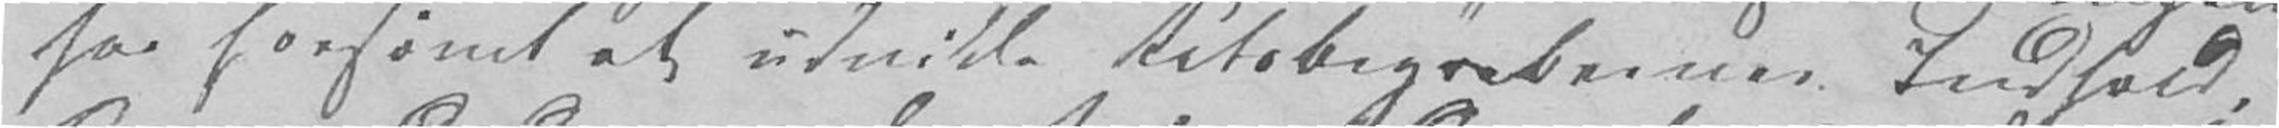har forsømt at udvikle Retsbegrebernes Indhold,
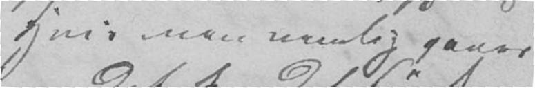Hvis man nemlig gaaer
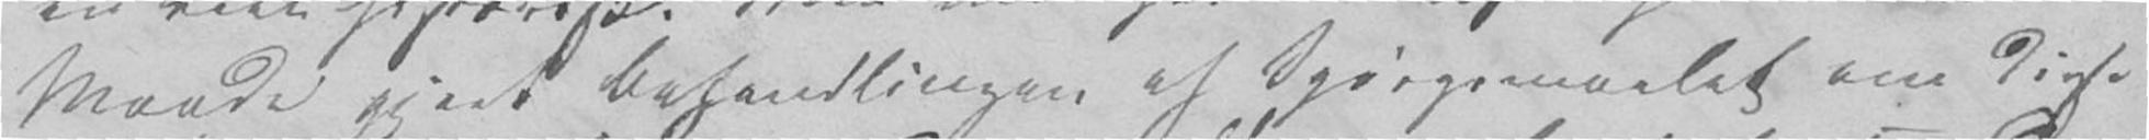Maade gjort Behandlingen af Spørgsmaalet om disse
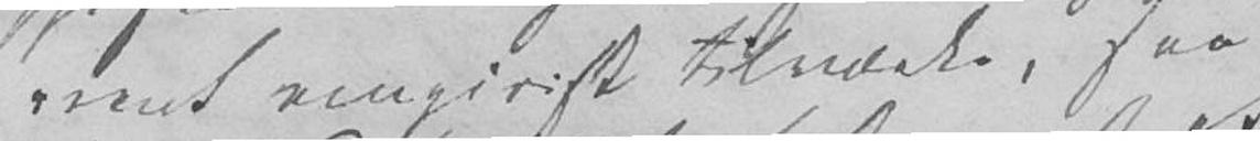rent empirisk tilværks, saa
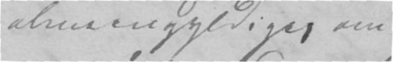almeengyldige, om
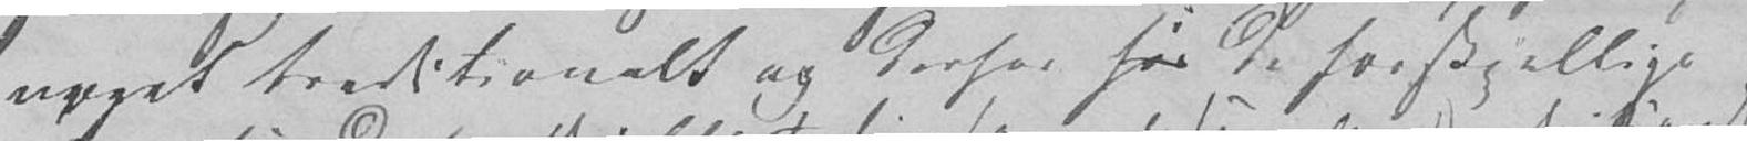noget traditionelt og derfor hos de forskjellige
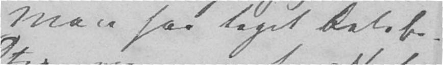Man har taget Retsbe-
